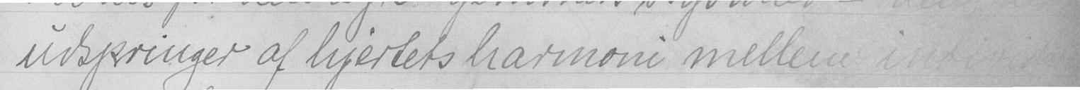udspringer af hjertets harmoni mellem mellem individerne
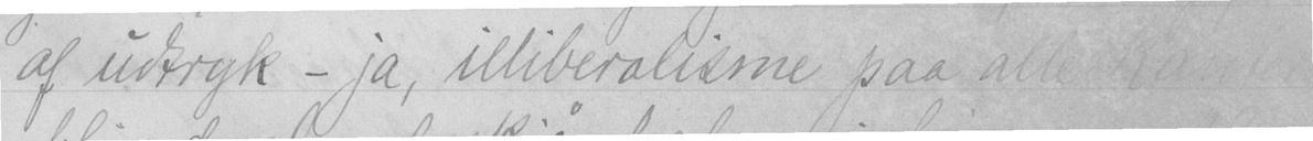af udtryk - ja, illiberalisme paa alle kante
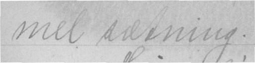mel sætning.
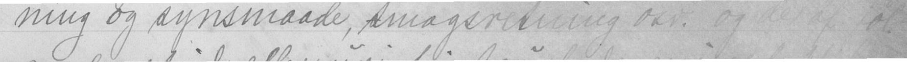ning og synsmaade, smagsretning osv. og deraf føl-
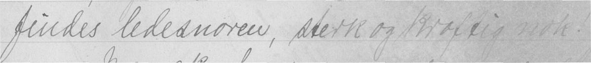findes ledesnoren, sterk og kraftig nok!
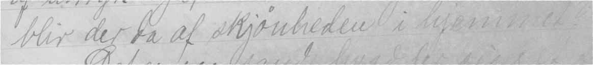blir der da af skjønheden i hjemmet
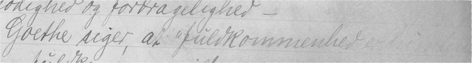Goethe siger, at "fuldkommenhed er hinsides
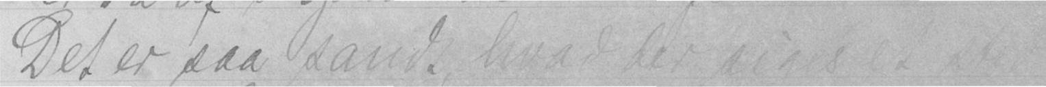Det er saa sandt, hvad der siges  at
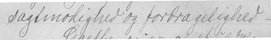sagtmodighed og fordragelighed -
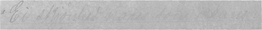"Ei skjønhed
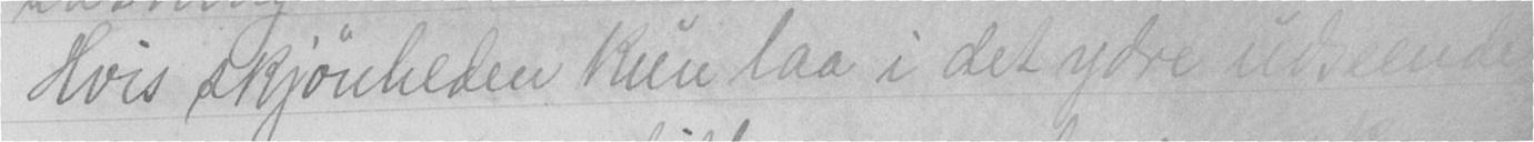Hvis skjønheden kun laa i det ydre udseende
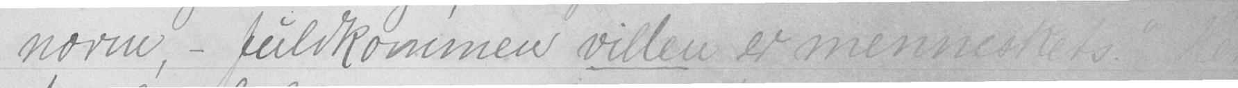norm, - fuldkommen villen er menneskets." Heri
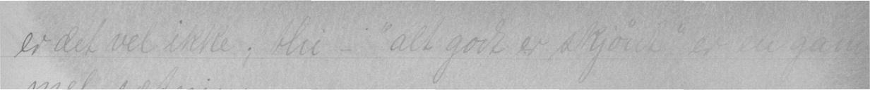er det vel ikke; this -"alt godt er skjønt" er en gam-
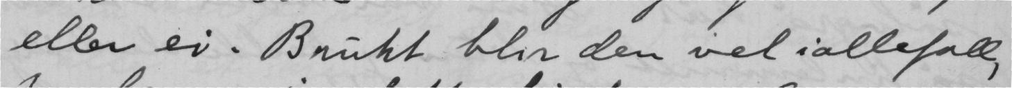eller ei. Brukt blir den vel iallefall,
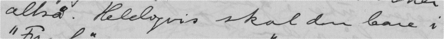altså. Heldigvis skal den bare i
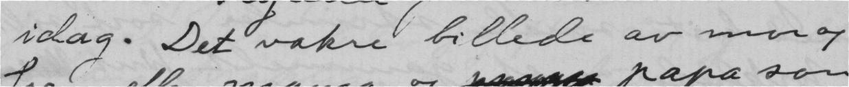idag. Det vakre billede av mor og
